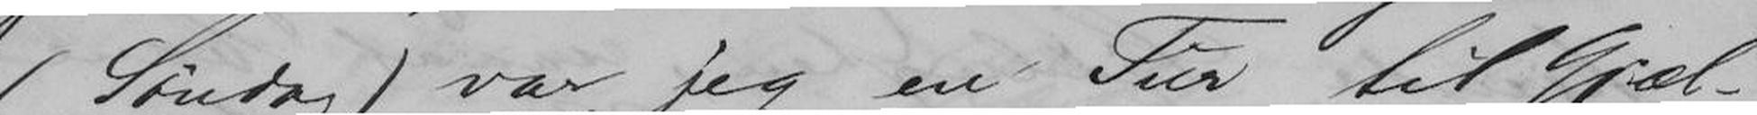(Søndag) var jeg en Tur til Gjæl-
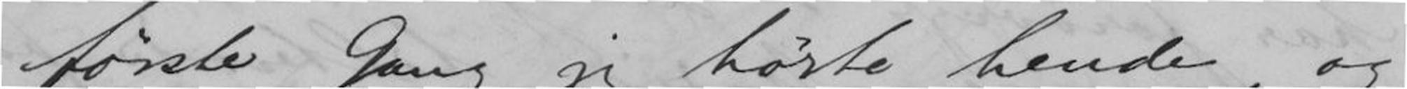første Gang jeg hørte hende, og
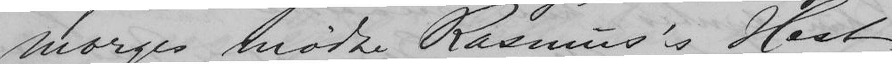morges mødte Rasmus's Hæst
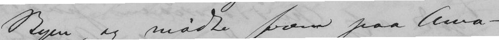Byen, og mødte frem paa Ama-
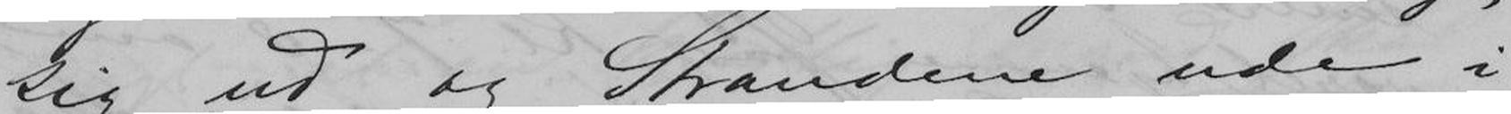sig ud og Strandene ude i
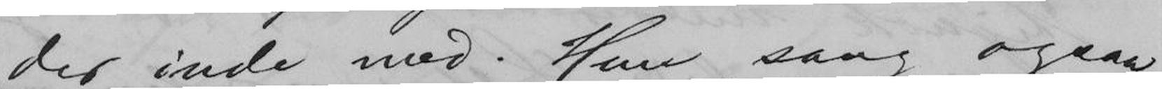der inde med. Hun sang ogsaa
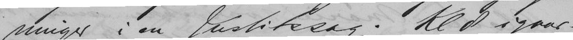ninger i en Justitssag. Kl 8 igaar-
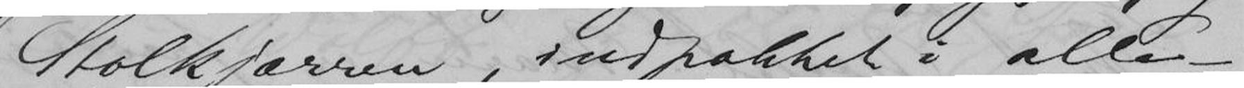Stolkjærren, indpakket i alle-
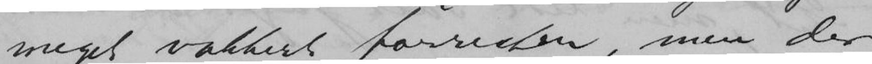meget vakkert forresten, men der
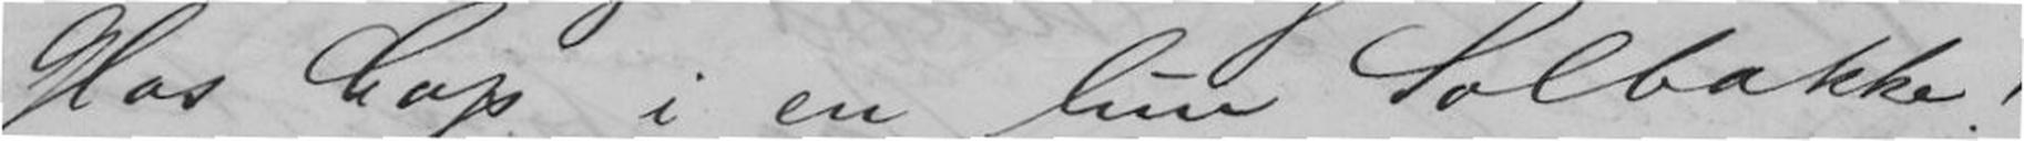Glas Cap i en lun Solbakke!
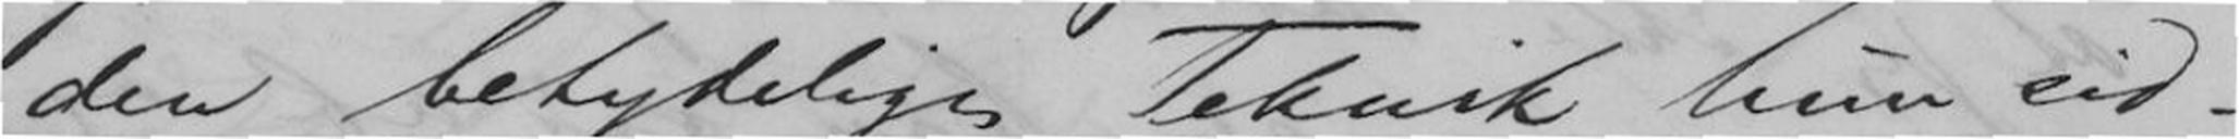den betydelige Teknik hun sid-
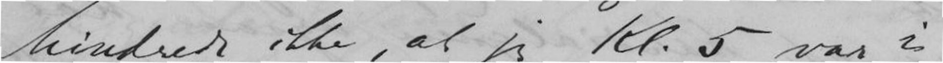hindrede ikke, at jeg Kl. 5 var i
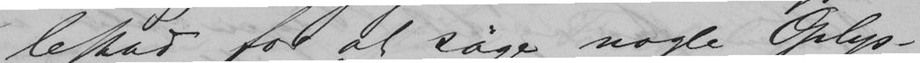lestad for at søge nogle Oplys-
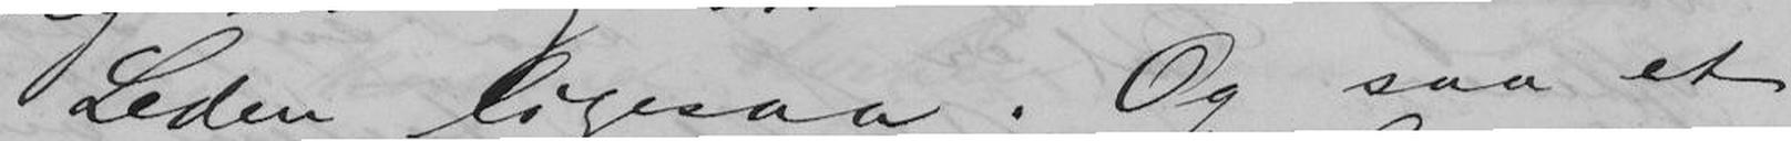Leden ligesaa. Og saa et
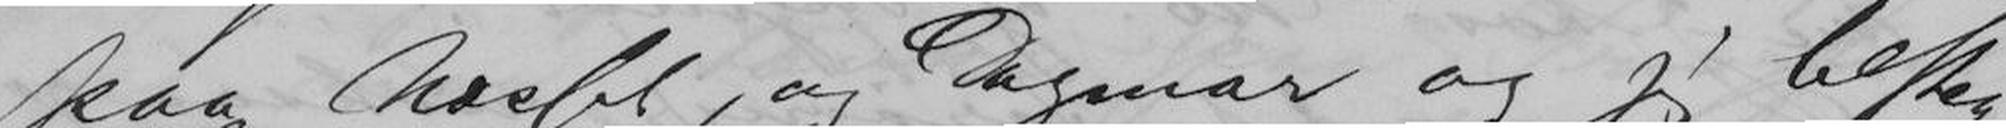paa Næsset, og Dagmar og jeg besteg
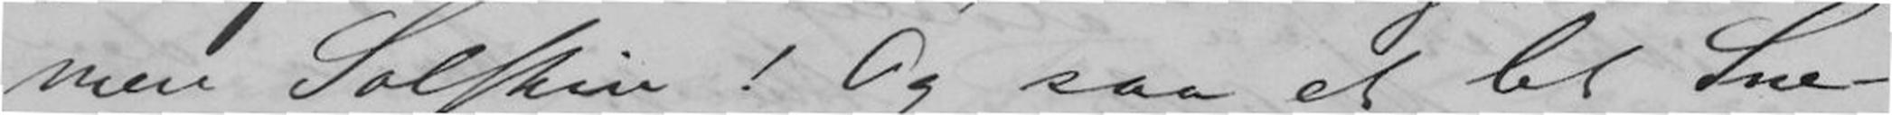men Solskin! Og saa et let Sne-
